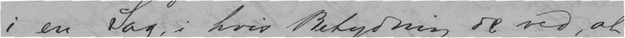i en Sag, i hvis Betydning de ved, at
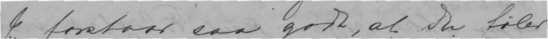Jeg forstaar saa godt, at Du føler
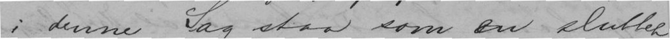i denne Sag staa som en sluttet
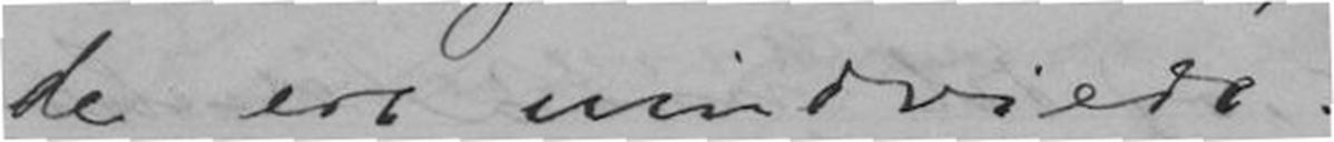de ere uindviede.
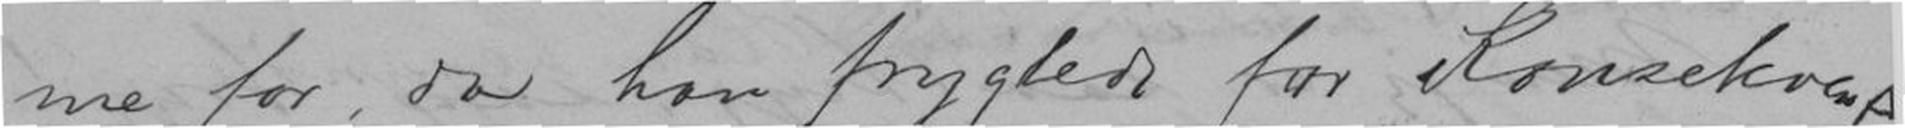me for, da han frygtede for Konsekvæn-
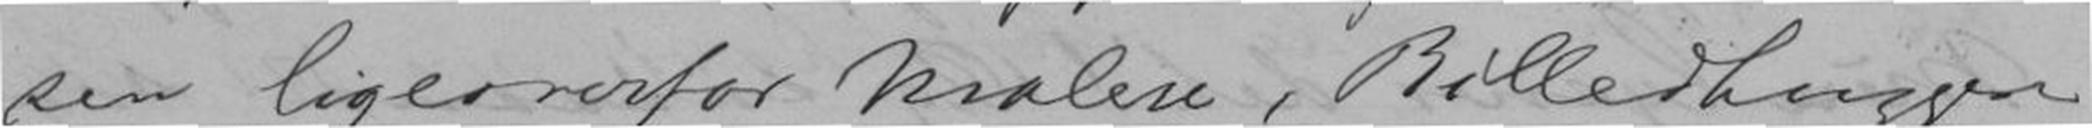sen ligeoverfor Malere, Billedhuggere
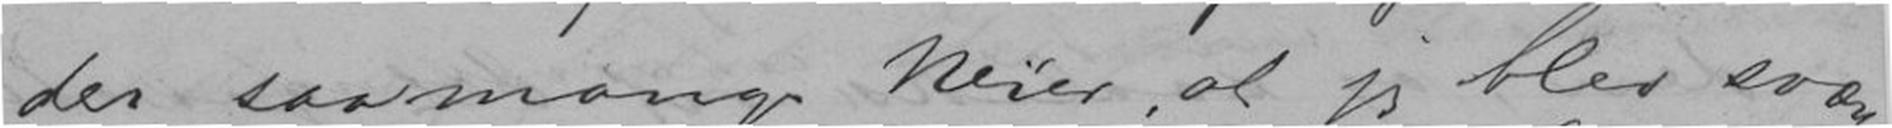der saamange Nei'er, at jeg blev svært
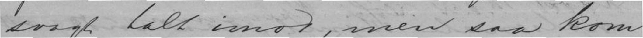svagt talt imod, men saa kom
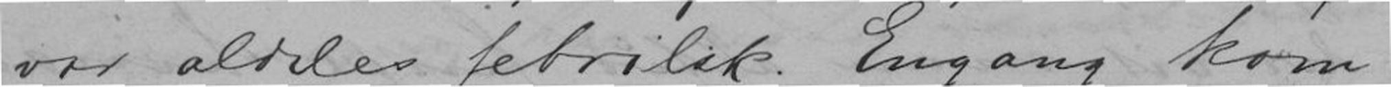var aldeles febrilsk. Engang kom
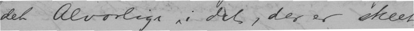det Alvorlige i det, der er skeet.
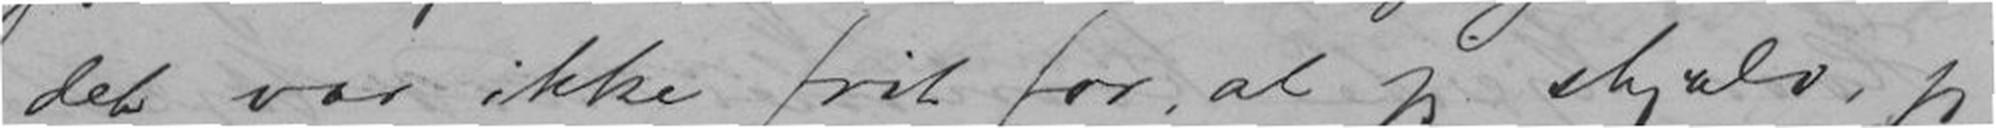det var ikke frit for at jeg skjalv, jeg
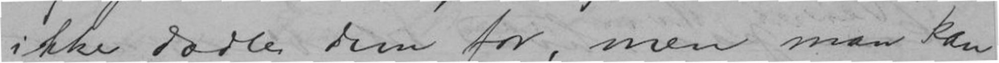ikke dadle dem for, men man kan
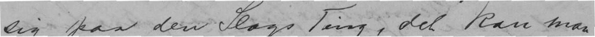sig paa den Slags Ting, det kan man
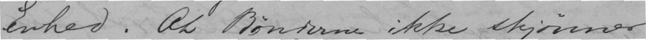Enhed. At Bønderne ikke skjønner
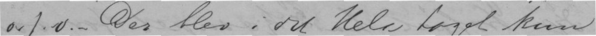o.s.v. Der blev i det Hele taget kun
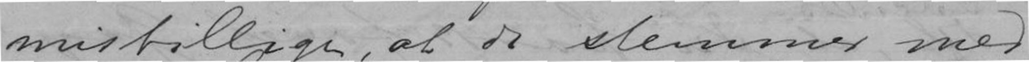misbillige, at de stemmer med
